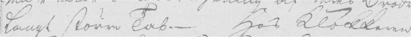langt større Tab. - Hos Klokkeren
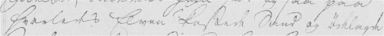hvorledes Elven kastede Sand og ødelagde
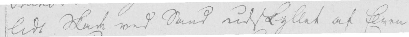lidt Skade ved Sand udskyllet af Elven
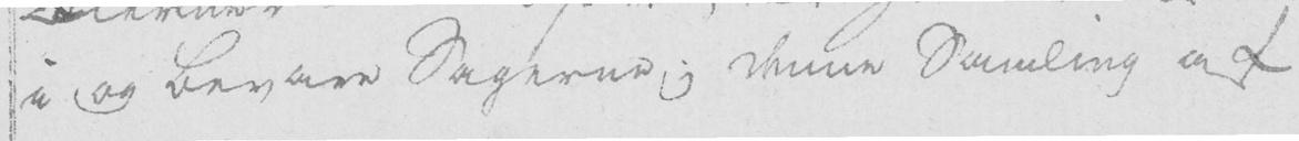i og bevare Sagerne; denne Samling af
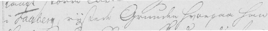i Faaberg, rystede Grunden hvorpaa han
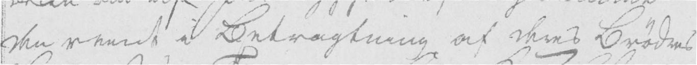den reent i Betragtning af deres Brødres
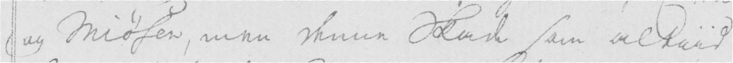og Miøsen, men denne Skade som altiid
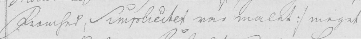Fromhed, Simplicitet var malet :/ meget
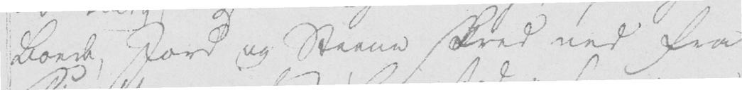boede, Jord og Steene skred ned fra
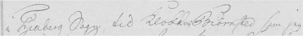i Faaberg Sogn, til Klokker Biørnsted som jeg
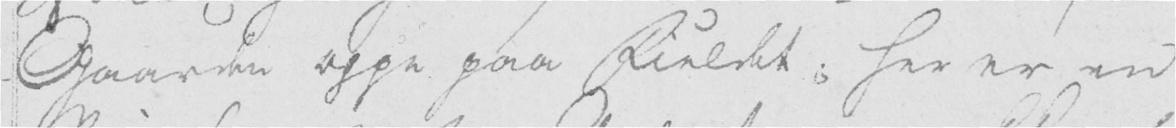Gaarden oppe paa Fieldet; her er en
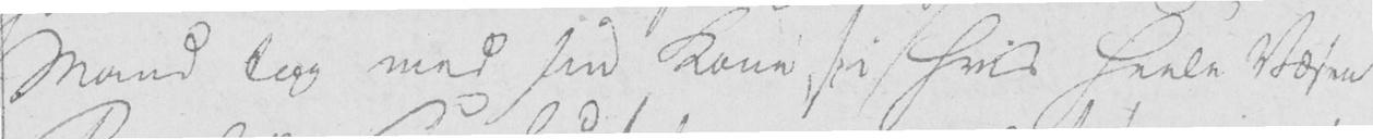Mand tog med sin Kone /: i hvis heele Væsen
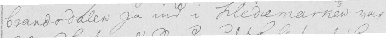brandsdalen Ja ind i Hedemarken var
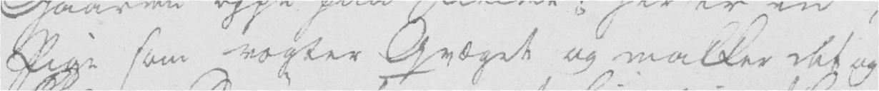Pige som vogter Qvæget og melker det og
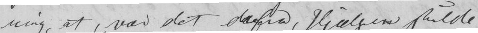ning, at, var det derfra, Hjælpen skulde
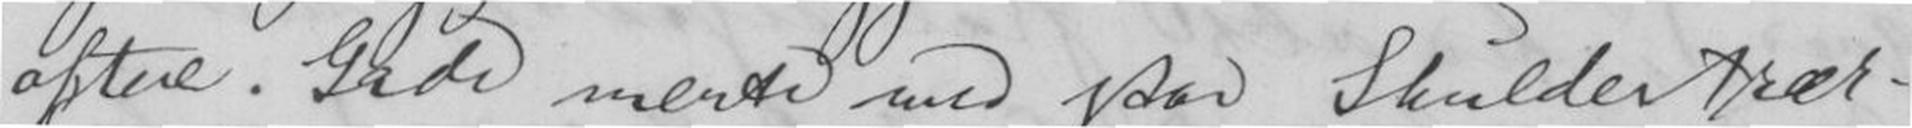oftere. Gade mente med stor Skuldertræk-
